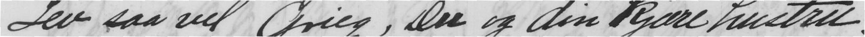Lev saa vel Grieg, Du og din kjære hustru!
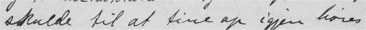skulde til at tine op igjen høres
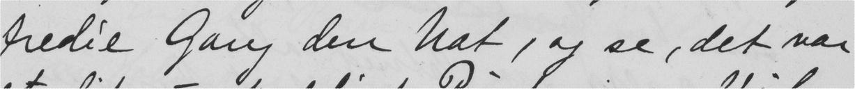tredie Gang den Nat, og se, det var
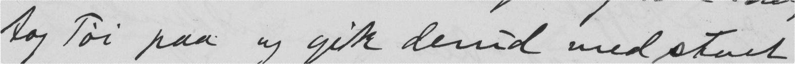tog Tøi paa og gik derud med stort
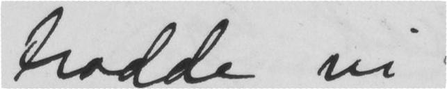trodde vi
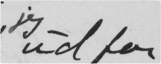ud for
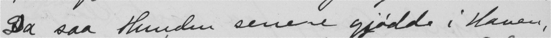Da saa Hunden senere gjødde i Haven,
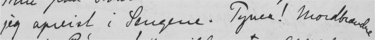jeg opreist i Sengene. Tyver! Mordbrændere
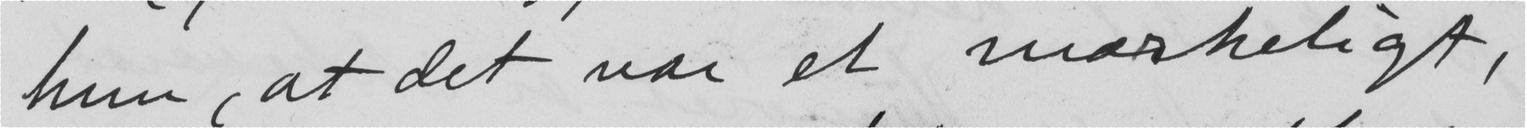hun, at det var et mærkeligt,
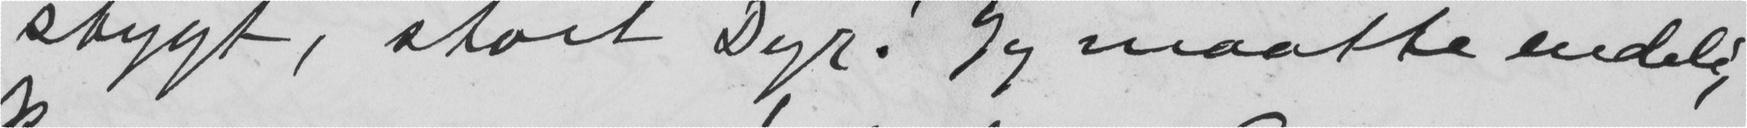stygt, stort Dyr! Jeg maatte endelig
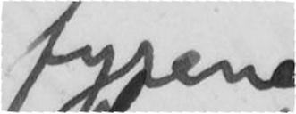fyrene
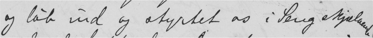og løb ind og styrtet os i Seng skjælvende
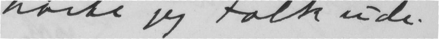hørde jeg Folk ude,
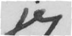jeg
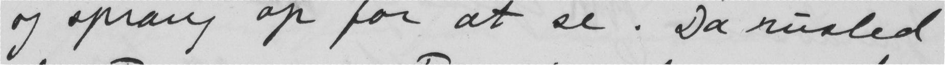og sprang op for at se. Da rusled
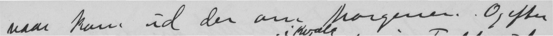vaar kom ud der om Morgenen. Og efter
